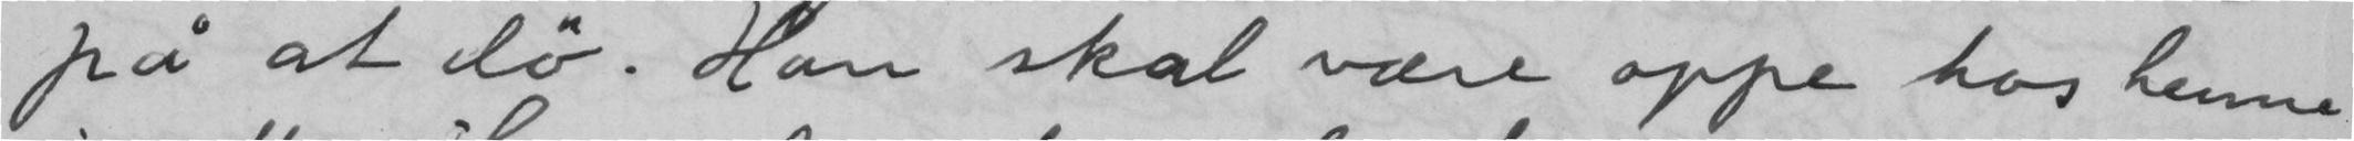på at dø. Han skal være oppe hos henne
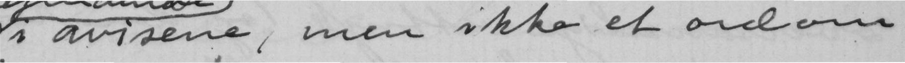i avisene, men ikke et ord om
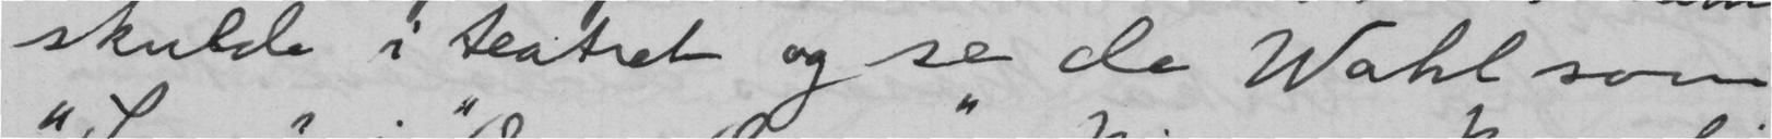skulde i teatret og se de Wahl som
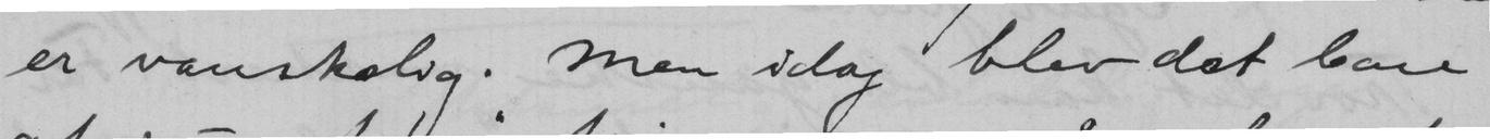er vanskelig. Men idag blev det bare
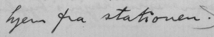hjem fra stationen.
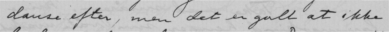danse efter, men det er galt at ikke
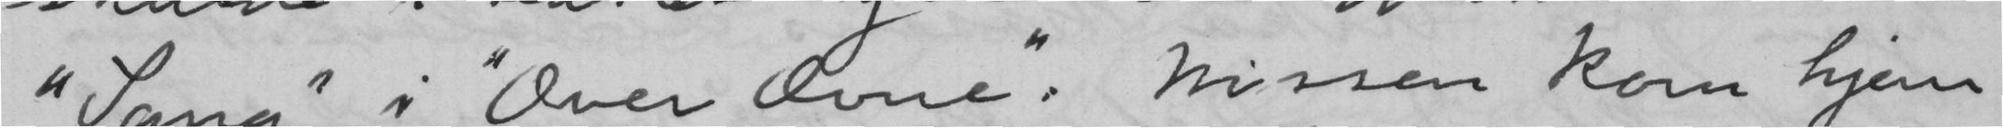"Sang" i "Over ævne". Nissen kom hjem
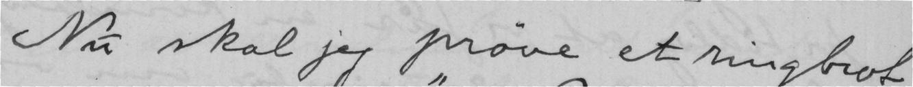Nu skal jeg prøve et ringbrot
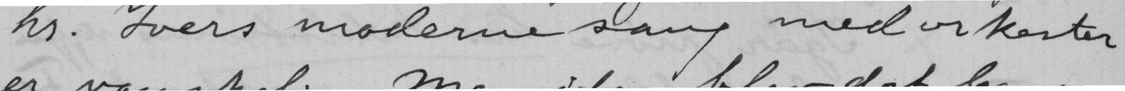hr. Ivars moderne sang med orkester
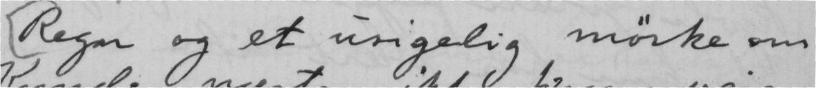Regn og et usigelig mørke om
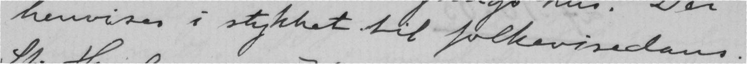henvises i stykket til folkevisedans.
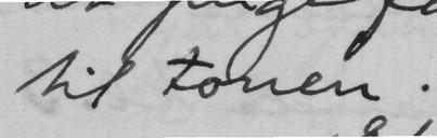til tonen.
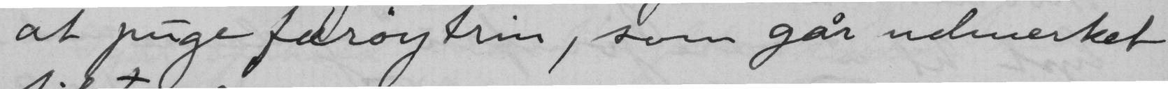at puge færøytrin, som går udmerket
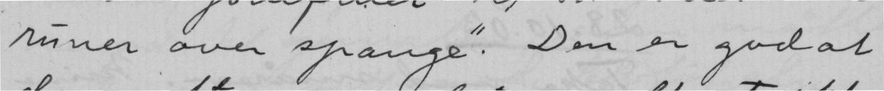runer over spange". Den er god at
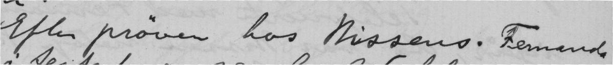Efter prøven hos Nissens. Fernanda
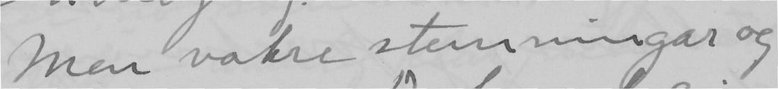Men vakre stemningar og
